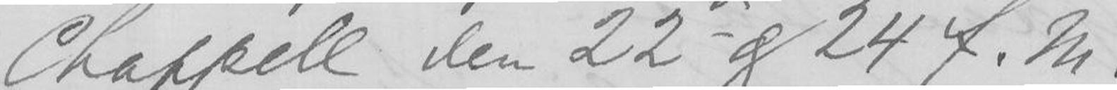Chappell den 22de og 24 f. M.
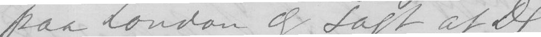paa London og sagt at De
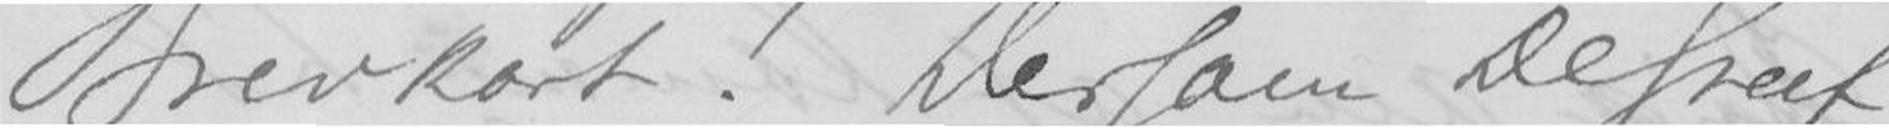Brevkort! Dersom De Greef
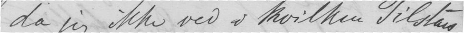da jeg ikke ved i hvilken Tilstan
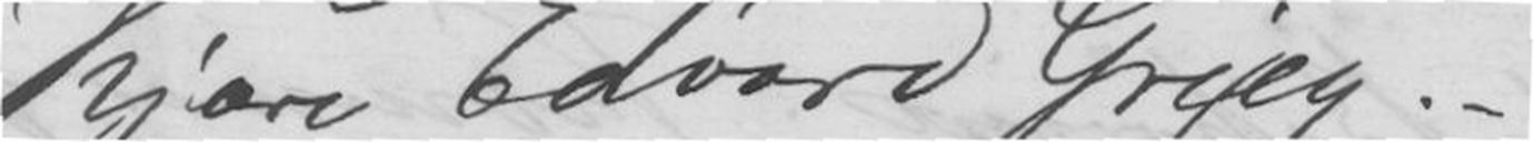Kjære Edvard Grieg. -
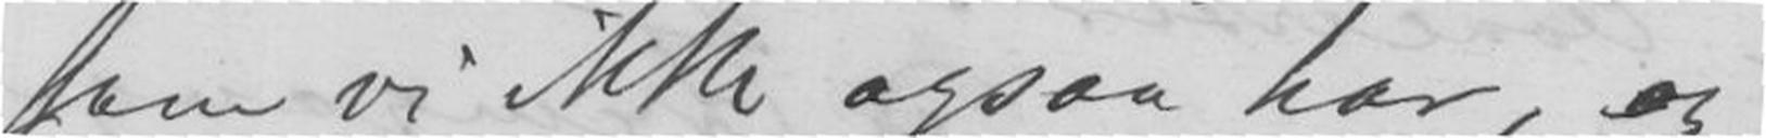som vi ikke ogsaa har, og
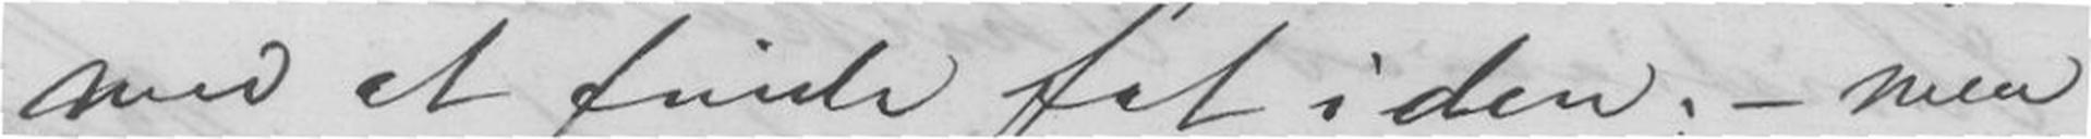med at finde fat i den; - men
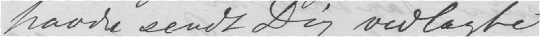havde sendt Dig vedlagte
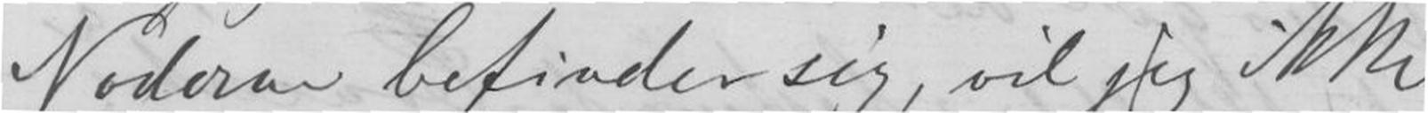Noderne befinder sig, vil jeg ikke
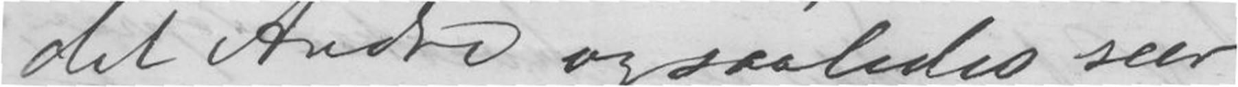det Andre og saaledes seer
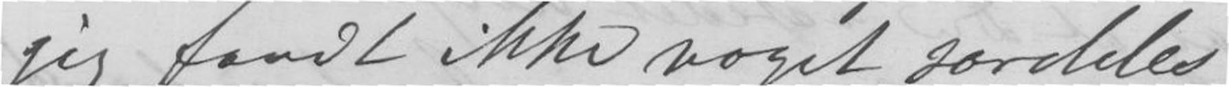jeg fandt ikke noget særdeles
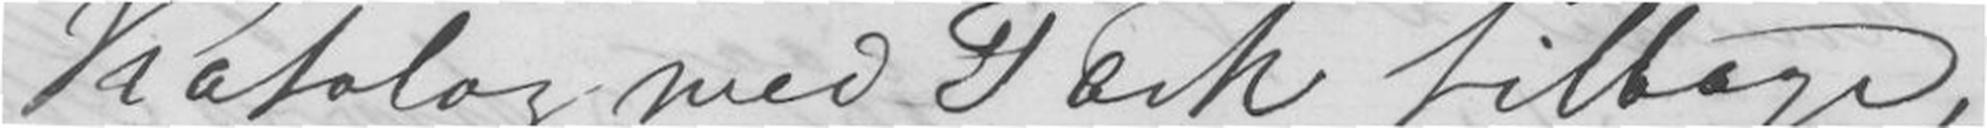Katalog med Tack tilbage,
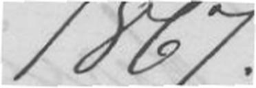1867.
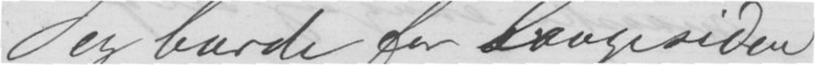Jeg burde for længe siden
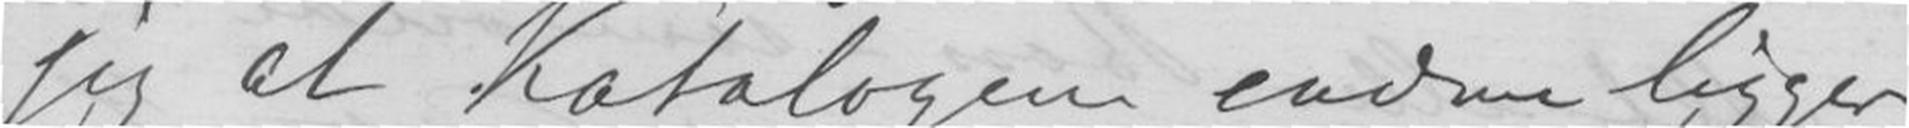jeg at Katalogen endnu ligger
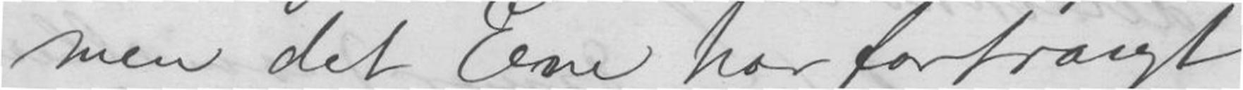men det Ene har fortrængt
